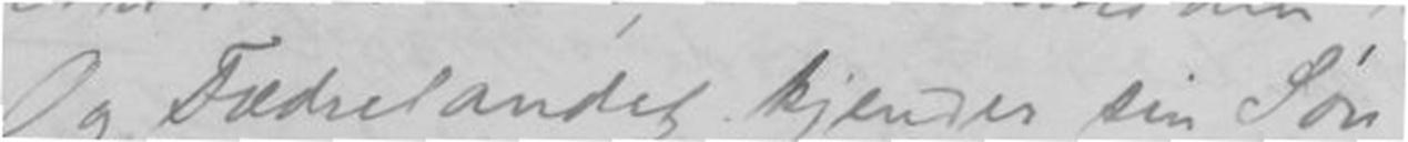Og Fædrelandet kjender sin Søn,
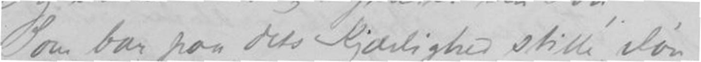Som bar paa dets Kjærlighed stille, døn
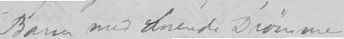Barm med anende Drømme,
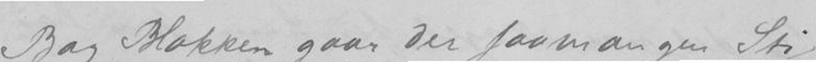Bag Blokken gaar der saamangen Sti,
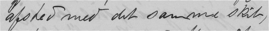afsted med det samme skib,
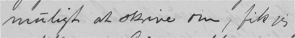muligt at skrive om, fik jeg
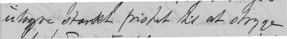uhyre stærkt fristet til at stryge
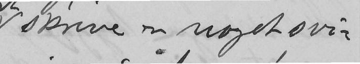skrive er noget svi-
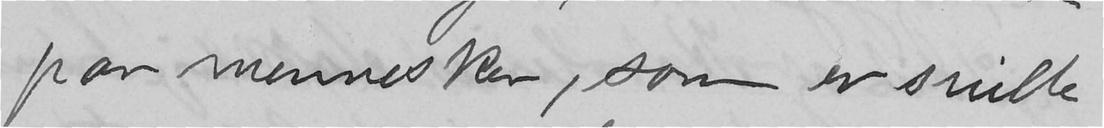par mennesker, som er snille
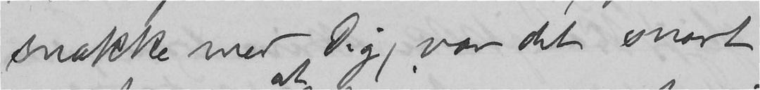snakke med Dig, var det snart
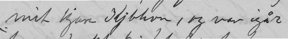mit kjære Kjbhvn, og var igår
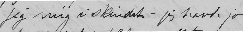jeg mig i skindet - jeg havde jo
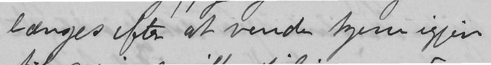længes efter at vende hjem igjen
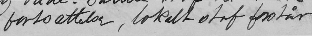fortsættelser, lokalt stof forstår
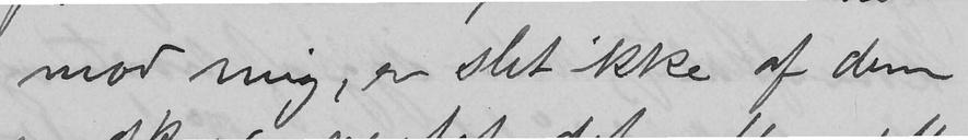mod mig, er slet ikke af dem
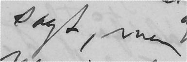sagt, men
